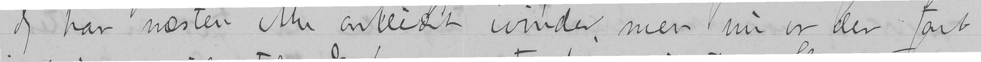Jeg har næsten ikke arbeidet ivinter, men nu er der fart
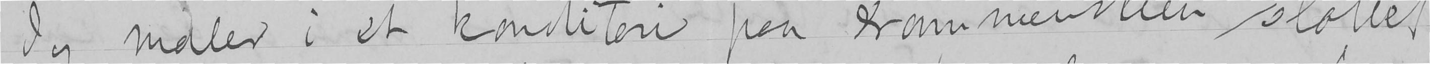Jeg maler i et konditori paa drammensveien slottet
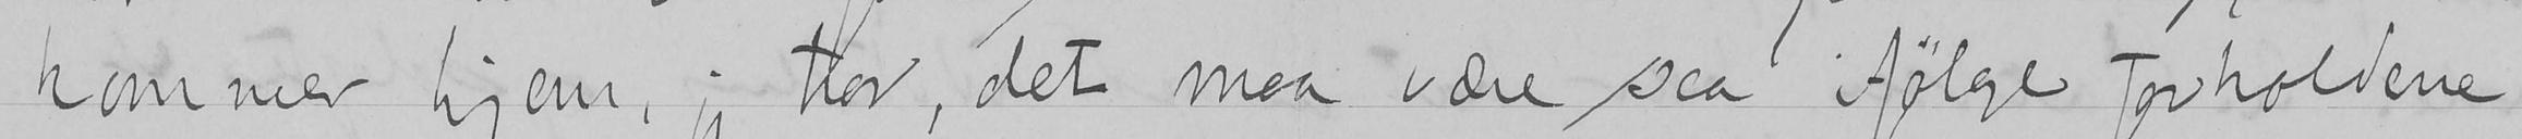kommer hjem, jeg tror, det maa være saa ifølge forholdene
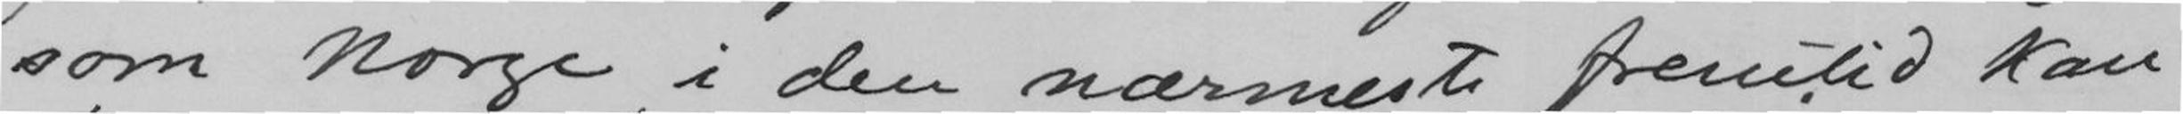som Norge i den nærmeste fremtid kan
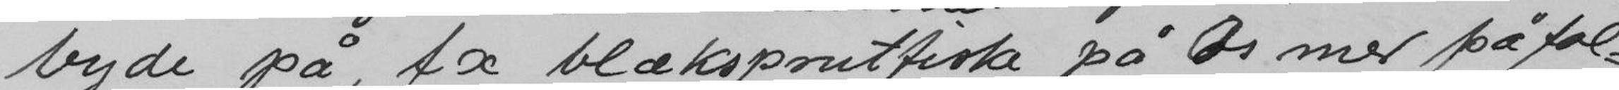byde på, f x blæksprutfisk på Os med påføl-
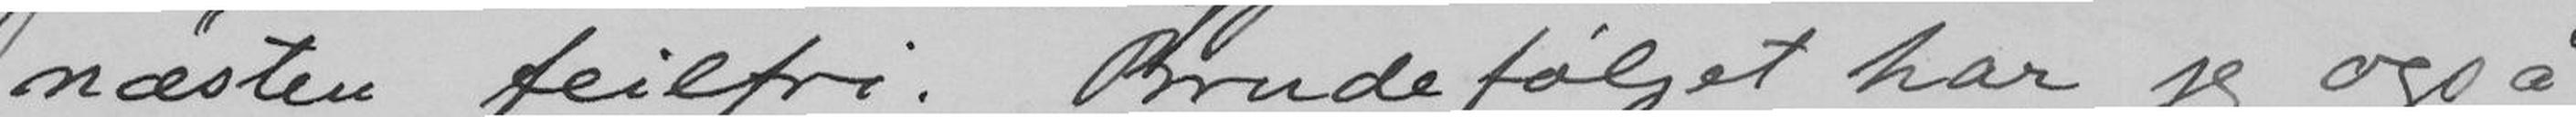næsten feilfri. Brudefølget har jeg også
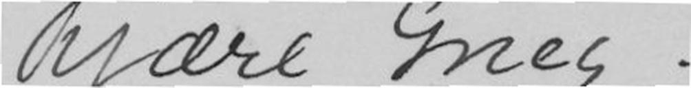Kjære Grieg!
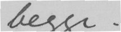begge.
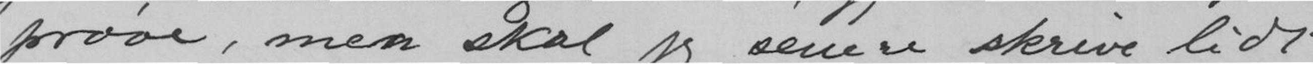prøve, men skal jeg senere skrive lidt
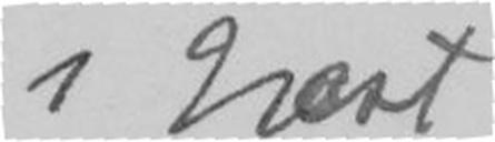i hast
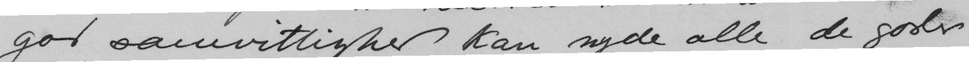god samvittighed kan nyde alle de goder
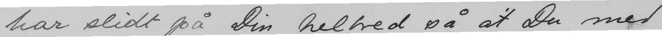har slidt på Din helbred så at Du med
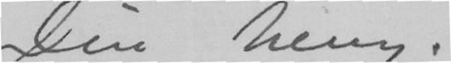Din heng.
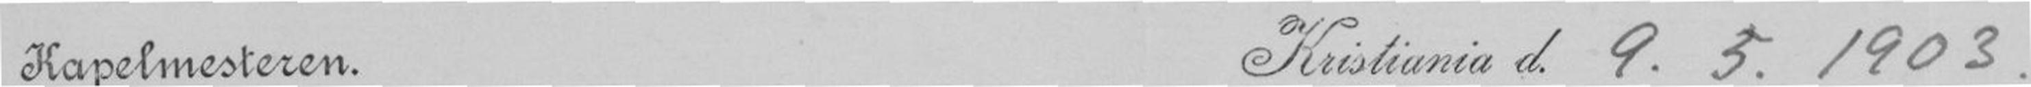Kapelmesteren. Kristiania d. 9.5.1903
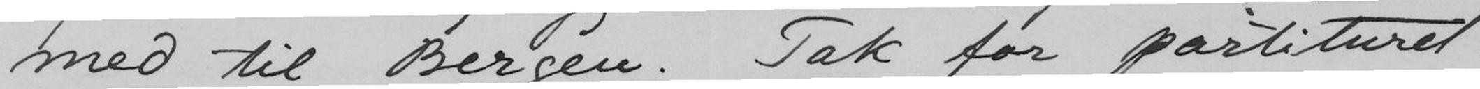med til Bergen. Tak for partituret.
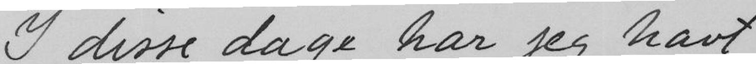I disse dage har jeg havt
